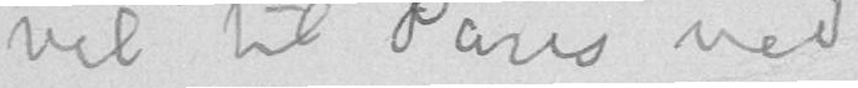vel til Paris ved
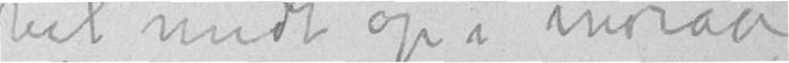vel midt op i moroa
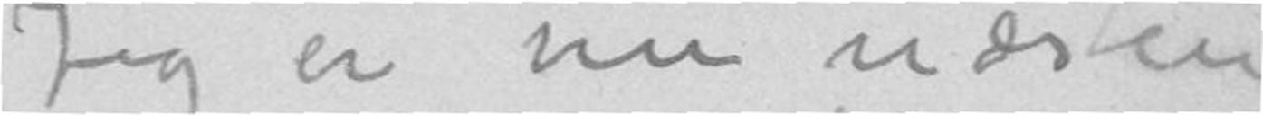Jeg er nu næsten
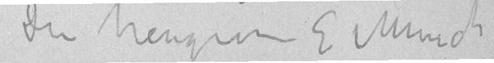Din hengivne E Munch
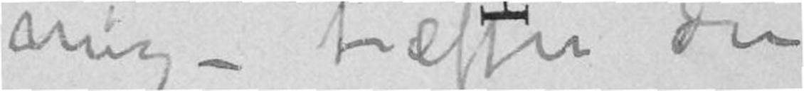mig – træffer du
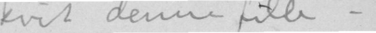kvit denne fille-
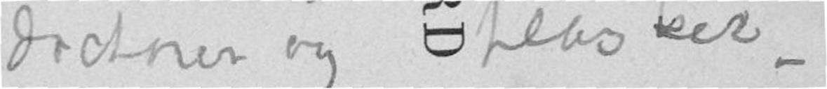doctorer og flasker –
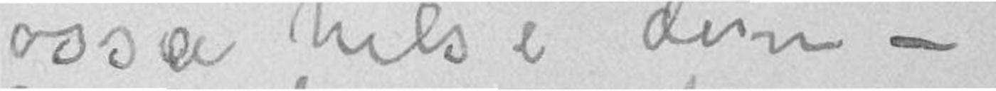osså hilse disse –
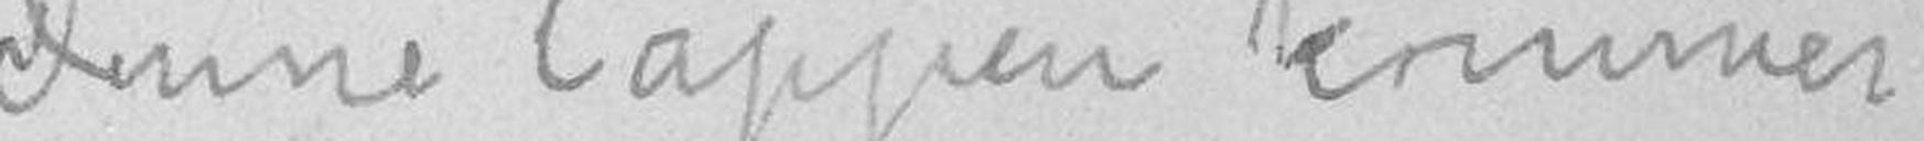Denne lappen kommer
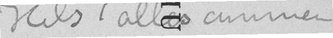Hils allesammen
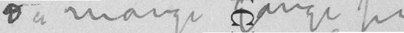så mange Gange fra
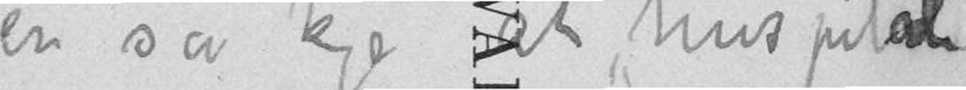er så kje af hospital
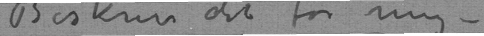Beskriv det for mig –
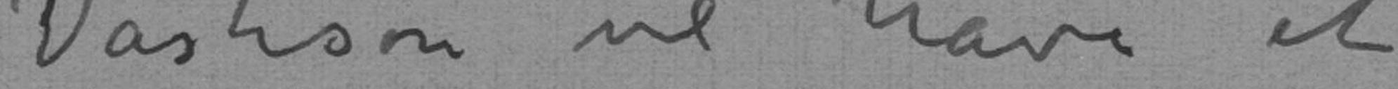Vasteson vil have et
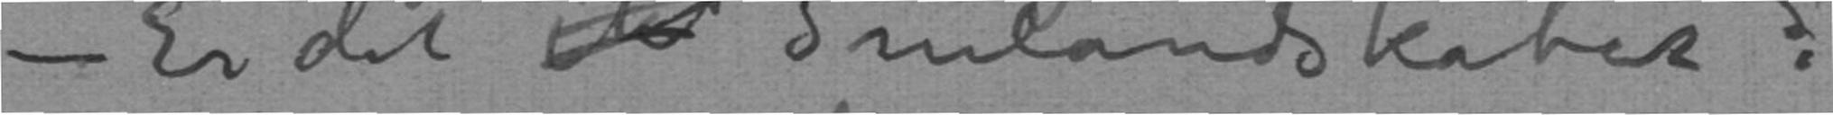– Er det ik Snelandskabet?
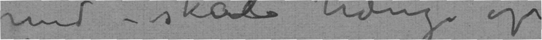med – skal hænge op
